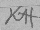KH
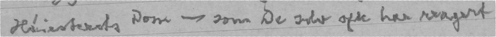Høiesterets Dom - som De selv ofte har reagert
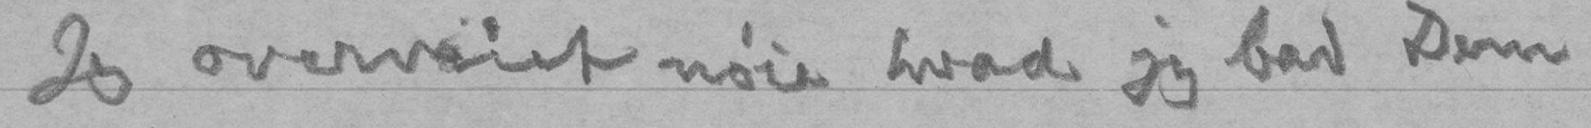Jeg overveiet nøie hvad jeg bad Dem
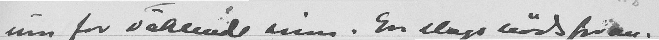um for våklende sinn. En slags nødsforan-
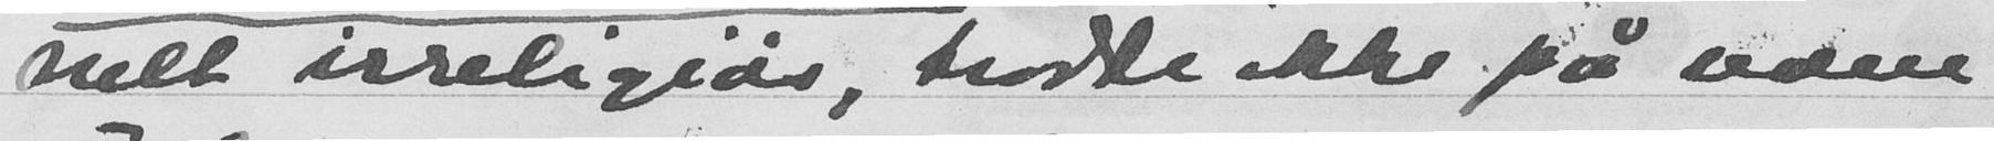vilt irreligiøs, trodde ikke på noen
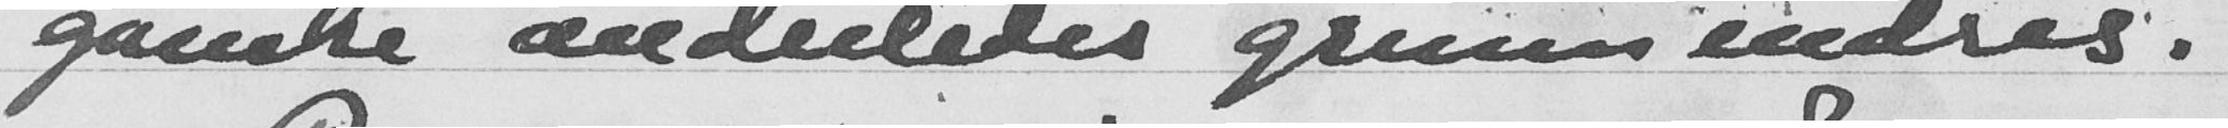ganske anderledes grunnendres.
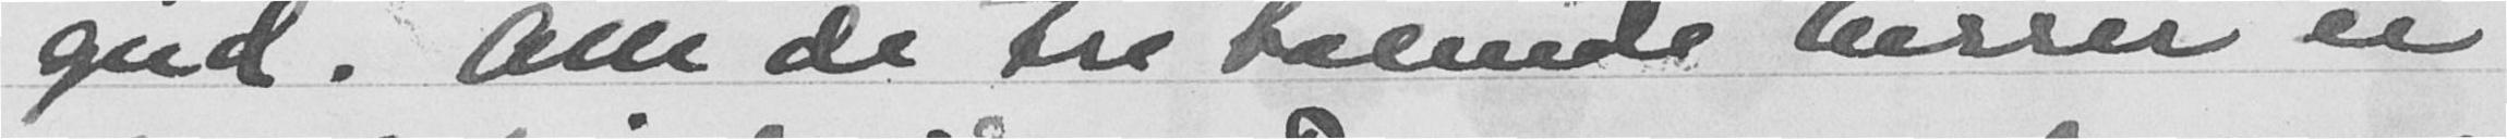gud. Alle de tre talende herrer er
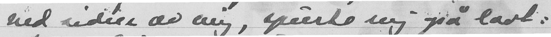ved siden av mig, spurte mig også lavt:
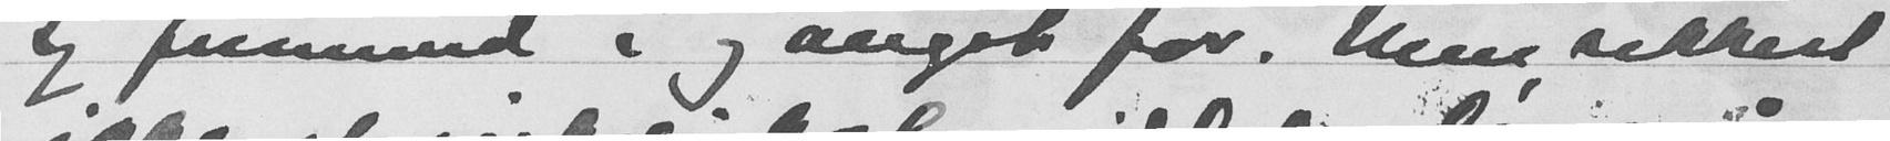sig fremmed i og angst for. Men sikkert
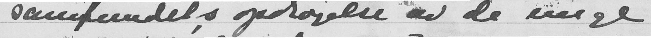samfundets opdragelse ev de unge
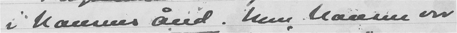i Nansens ånd. Men Nansen var
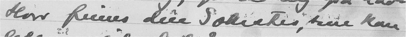Hvor finnes dere Sokrater, som kan
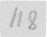118.
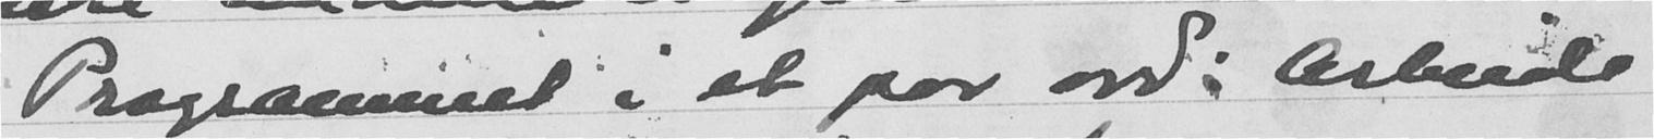Programmet i et par ord; arbeide
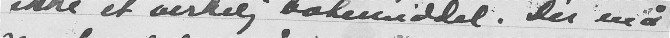ikke et virkelig botemiddel. Der må
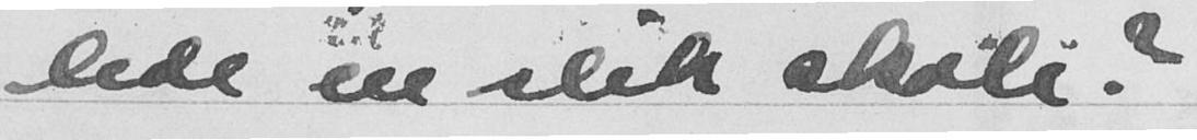lede en slik skole?
